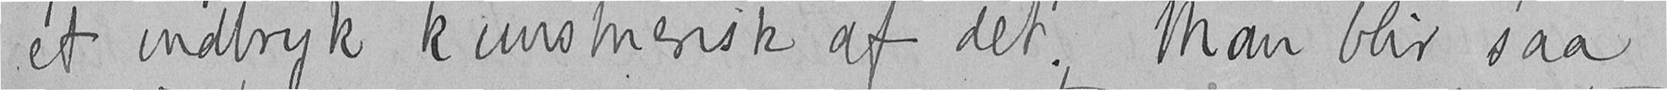et indtryk kunstnerisk af det. Man blir saa
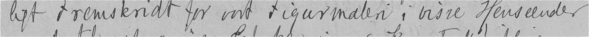ligt Fremskridt for vort Figurmaleri i visse Henseender
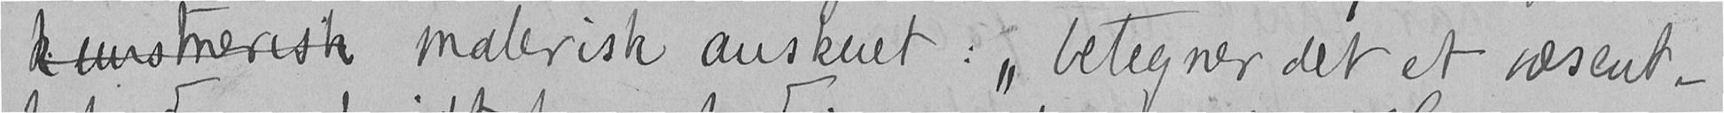kunstnerisk malerisk anskuet: "betegner det et væsent-
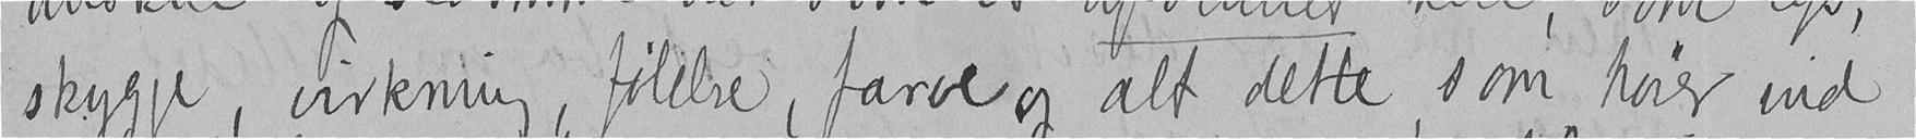skygge, virkning, følelse, farve og alt dette, som hører med
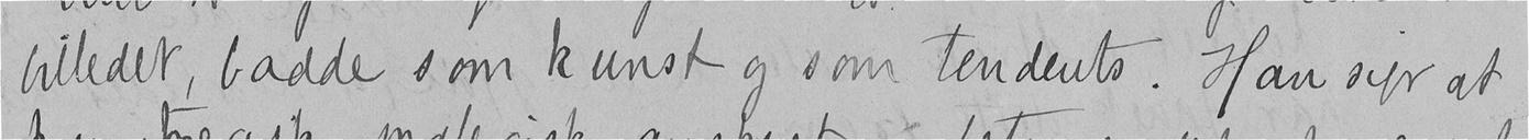billedet, baade som kunst og som tendents. Han siger at
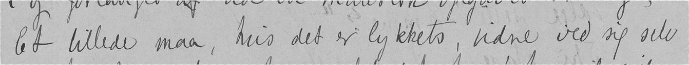Et billede maa, hvis det er lykkets, vidne ved sig selv
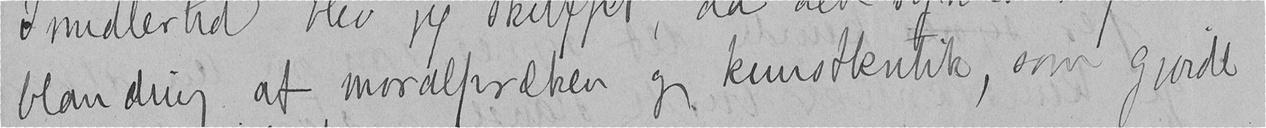blanding af moralpræken og kunstkritik, som gjorde
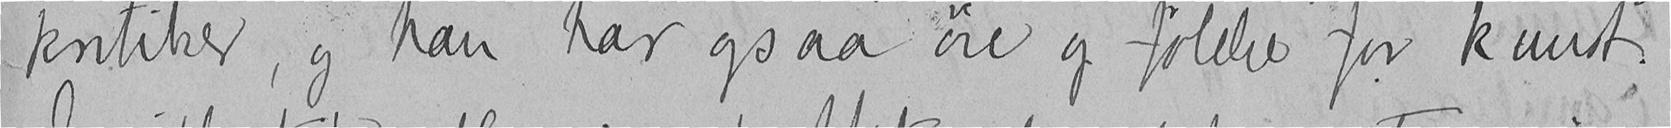kritiker, og han har ogsaa øie og følelse for kunst.
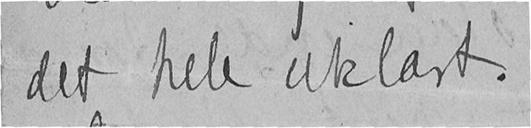det hele uklart.
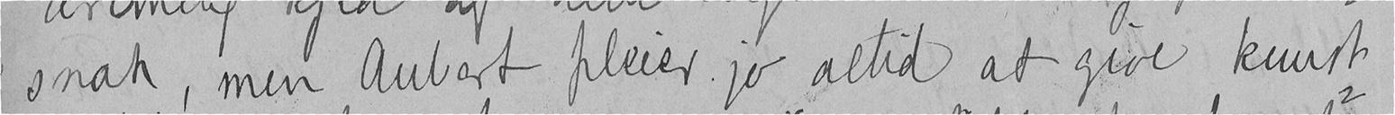snak, men Aubert pleier jo altid at give kunst-
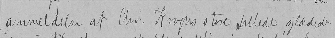ammeldelse af Chr. Kroghs store billede, glædede
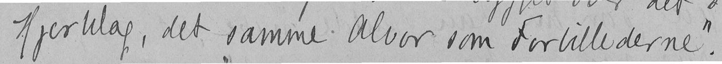Hjertelag, det samme Alvor som Forbillederne".
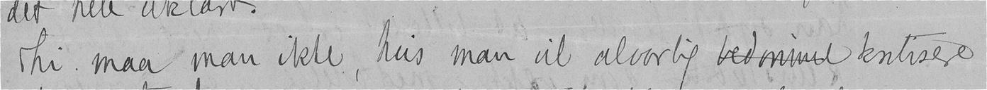Thi maa man ikke, hvis man vil alvorlig bedømme kritisere
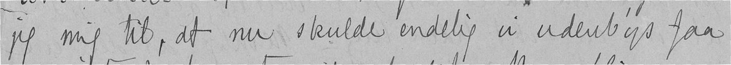jeg mig til, at nu skulde endelig vi udenbys faa
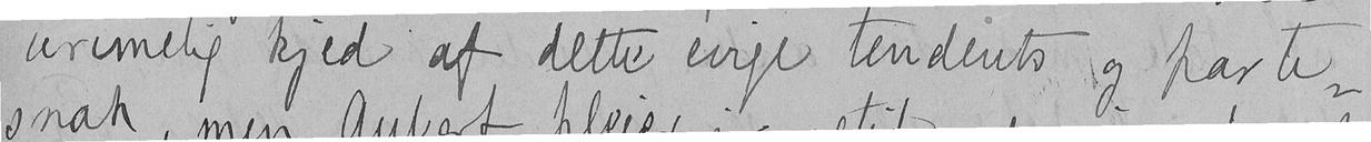urimelig kjed af dette evige tendents og parti-
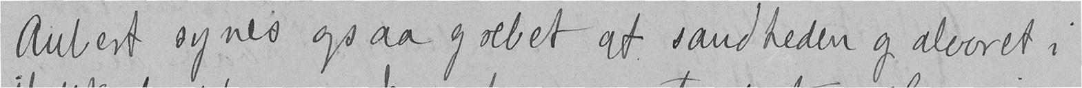Aubert synes ogsaa grebet af sandheden og alvoret i
